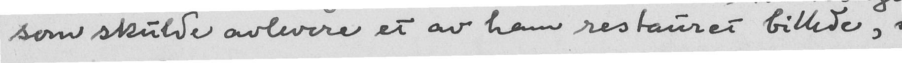som skulde avlevere et av ham restauret billede,
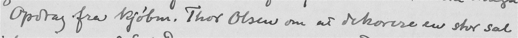Opdrag fra kjøbm. Thor Olsen om at dekorere en stor sal
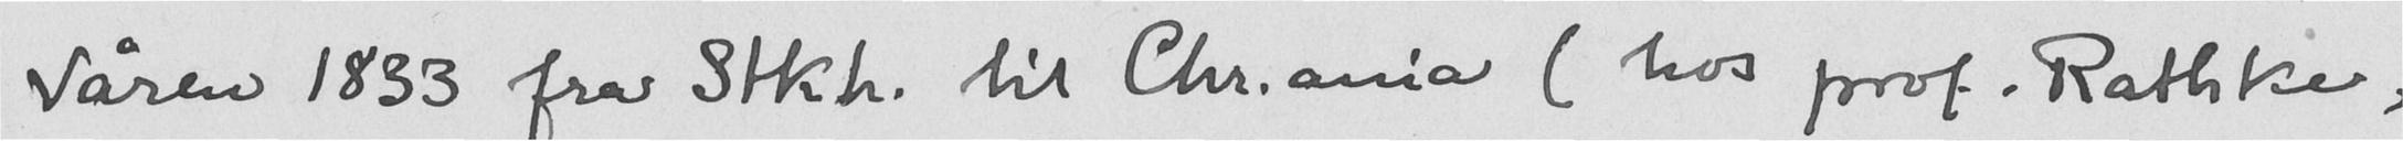Våren 1833 fra Stkh. til Chr.ania (hos prof. Rathke,
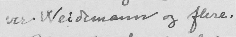ver Weidemann og flere.
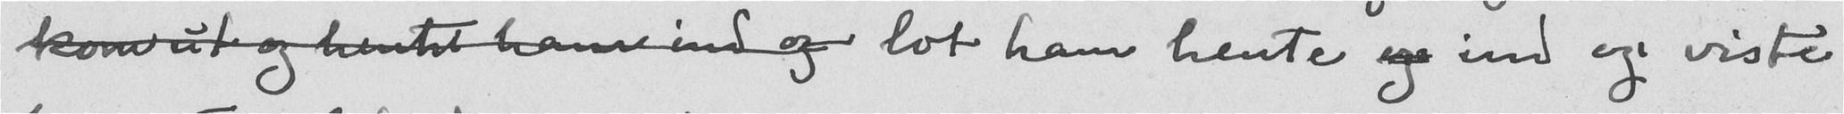kom ut og hentet ham ind og lot ham hente og ind og viste
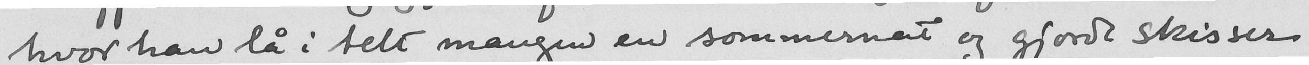hvor han lå i telt mangen en sommernat og gjorde skisser
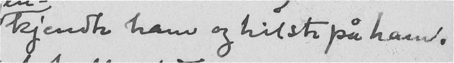kjendte ham og hilste på ham.
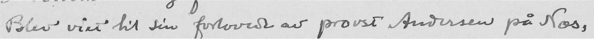Blev viet til sin forlovede av provst Andersen på Næs,
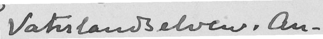Vaterlandselven. An-
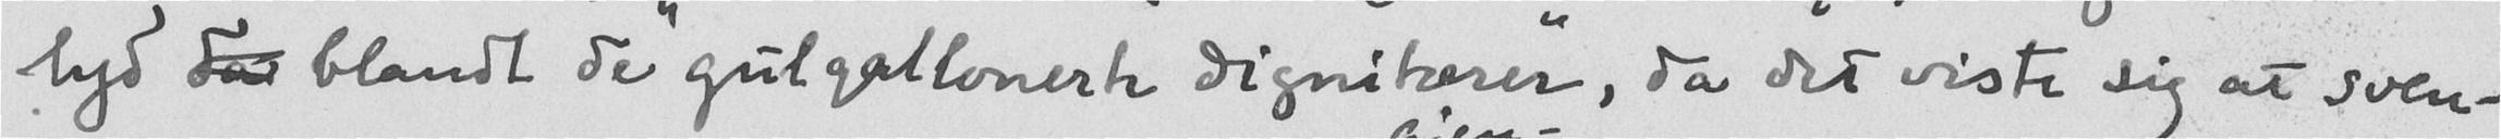lyd da blandt de "gulgallonerte dignitærer", da det viste sig at sven-
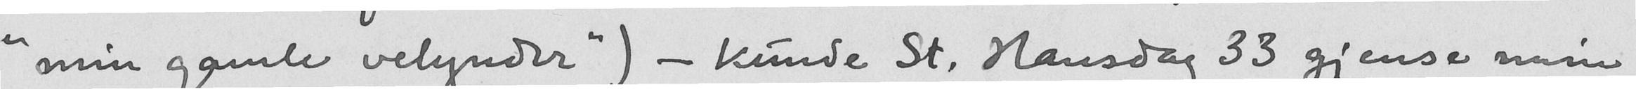min gamle velynder) - kunde St. Hansdag 33 gjense min
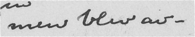men blev av-
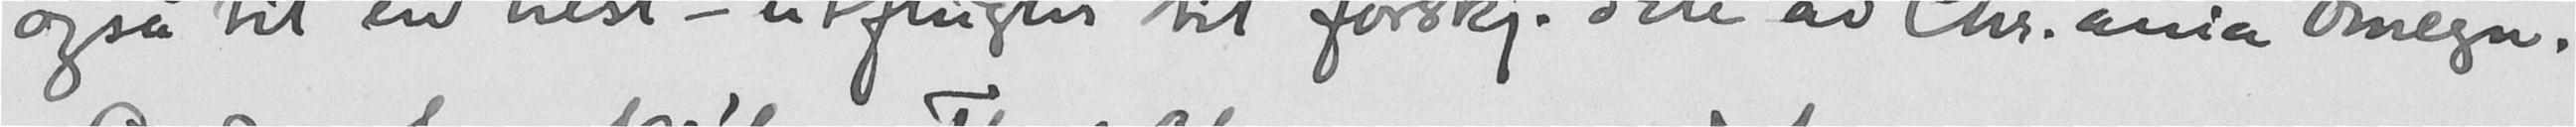også til en hest - utflugter til forskj. dele av Chr.ania omegn.
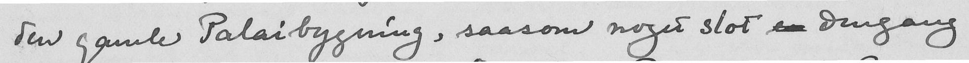den gamle Palaibygning, saasom noget slot en dengang
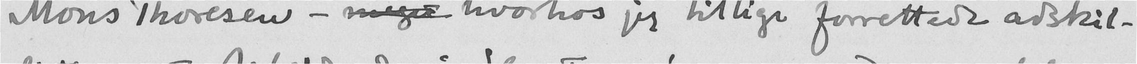Mons Thoresen - meget hvorhos jeg tillige forrettede adskil-
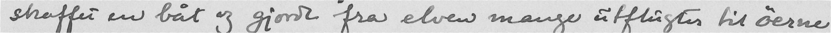skaffet en båt og gjorde fra elven mange utflugter til øerne
